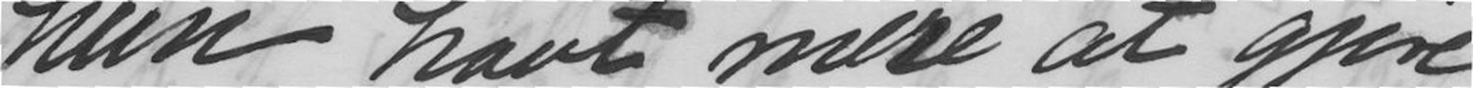hun havt mere at gjøre
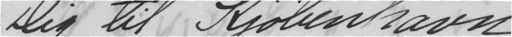Dig til Kjøbenhavn
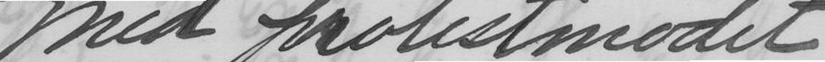Med protestmødet
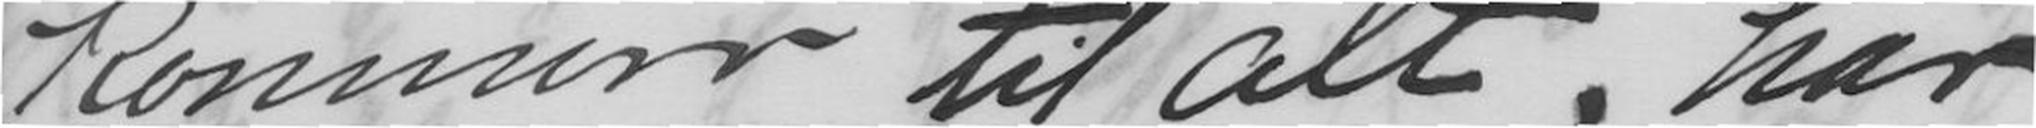kommer til alt, har
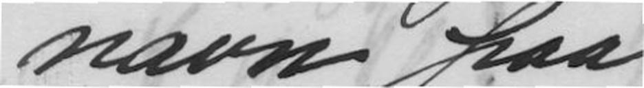navn paa
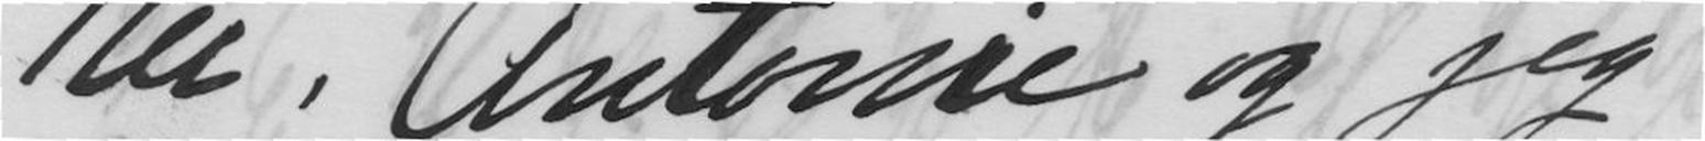Nei, Antonie og jeg
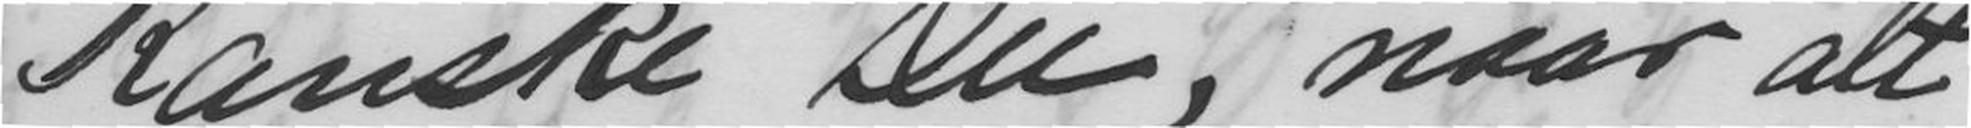Kanske Du, naar alt
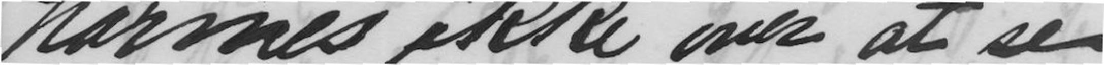harmes ikke over at se
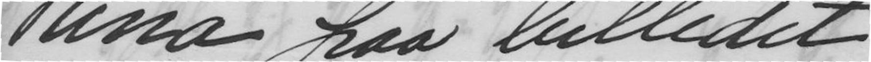Nina paa billedet.
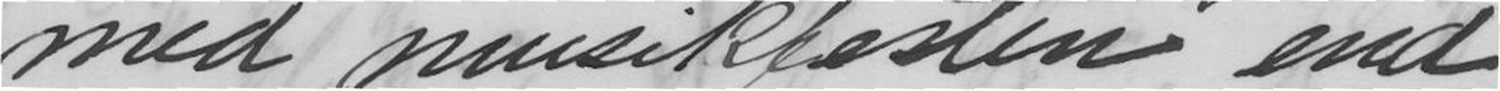med musikfesten end
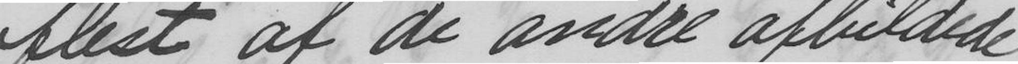flest af de andre afbildede.
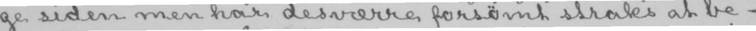ge siden men har desværre forsømt straks at be-
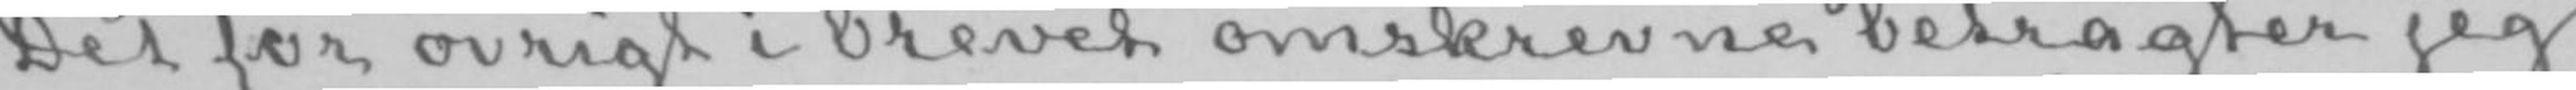Det for øvrigt i brevet omskrevne betragter jeg
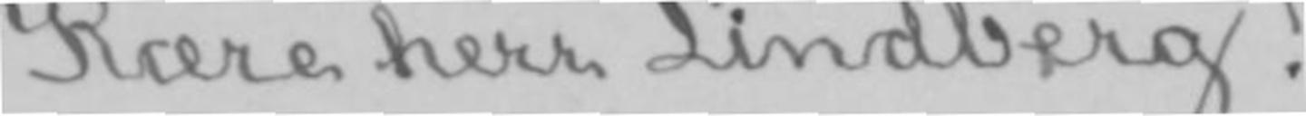Kære herr Lindberg!
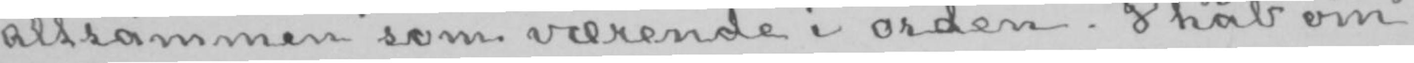altsammen som værende i orden. I håb om
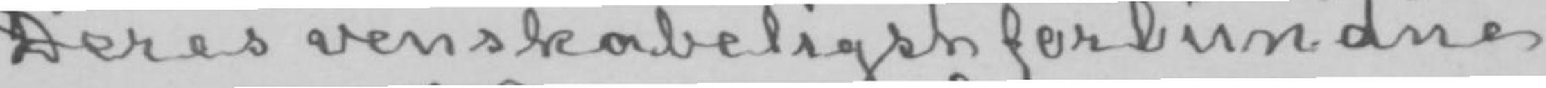Deres venskabeligst forbundne
